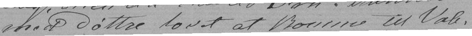med Døttre lovet at komme til Vale-
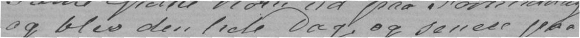og blev den hele Dag og senere paa
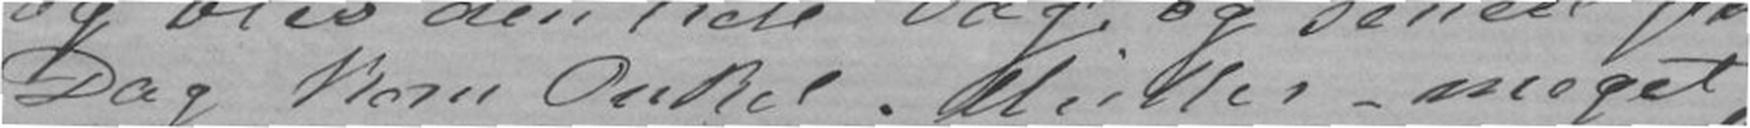Dag kom Onkel Müller - meget
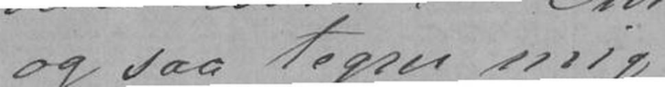og saa tegne mig
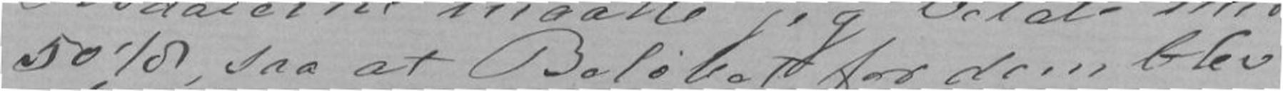50 1/8, saa at Beløbet for dem blev
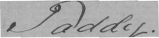Paddy.
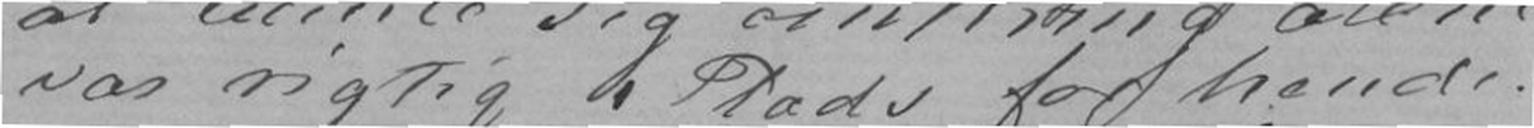var rigtig Plads for hende.
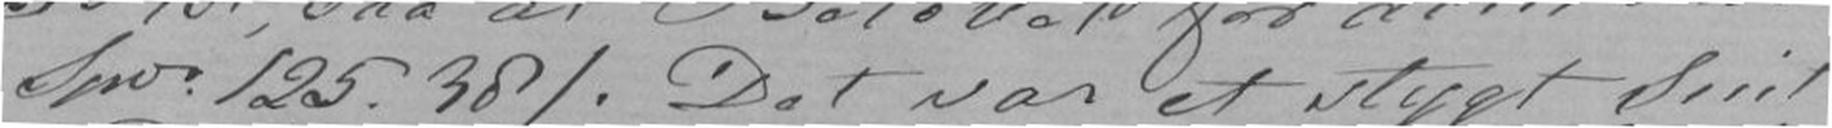Spd.. 125.38/. Det var et stygt Snit
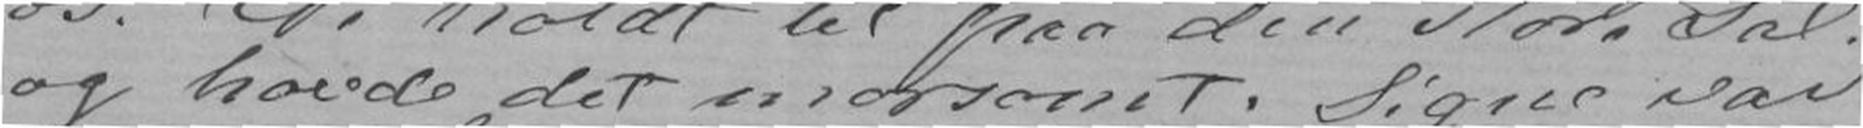og havde det morsomt. Signe var
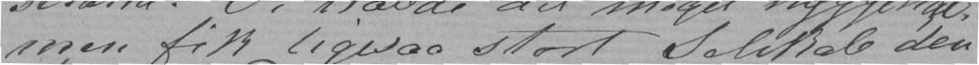men fik ligesaa stort Selskab den
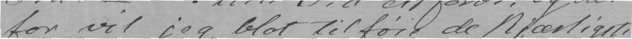for vil jeg blot tilføie de kjærligste
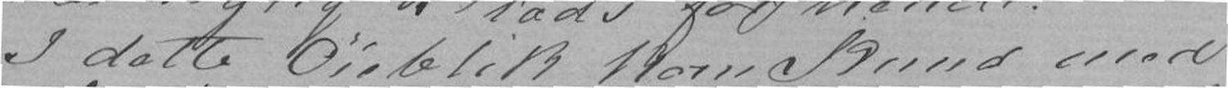I dette Øieblik kom Knud med
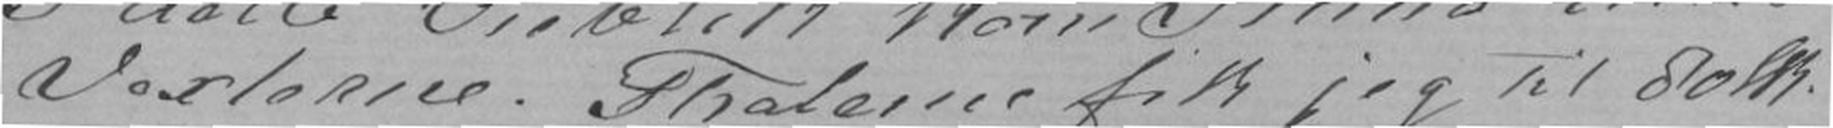Vexlerne. Thalerne fik jeg til 80 Stk.
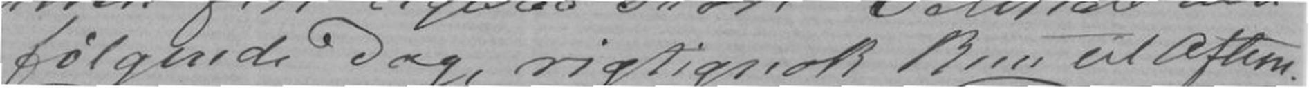følgendee Dag, rigtignok kun til Aftem.
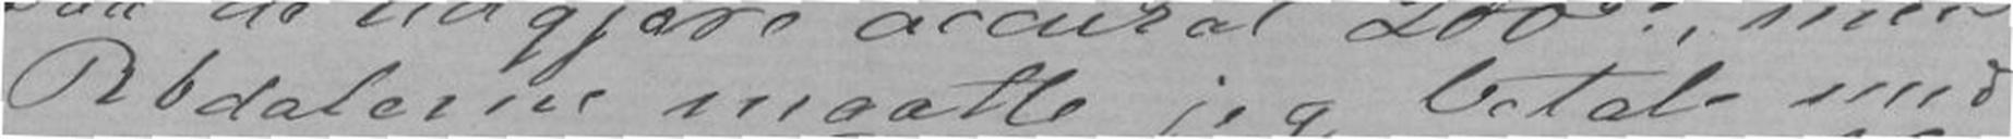R.dalerne maatte jeg betale med
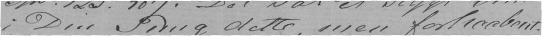i Din Pung dette, men forhaabent-
